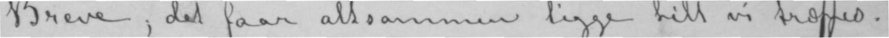Breve; det faar altsammen ligge till vi træffes.
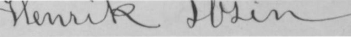Henrik Ibsen.
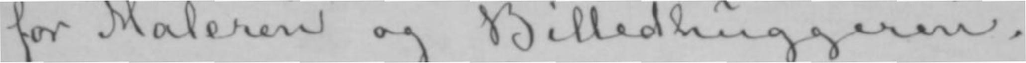for Maleren og Billedhuggeren.
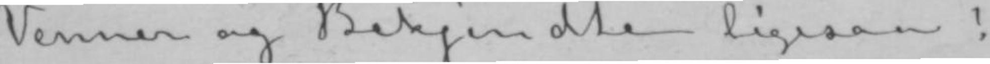Venner og Bekjendte ligesaa!
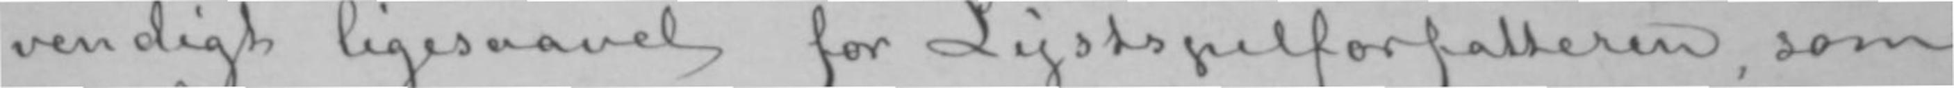vendigt ligesaavel for Lystspilforfatteren, som
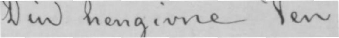Din hengivne Ven
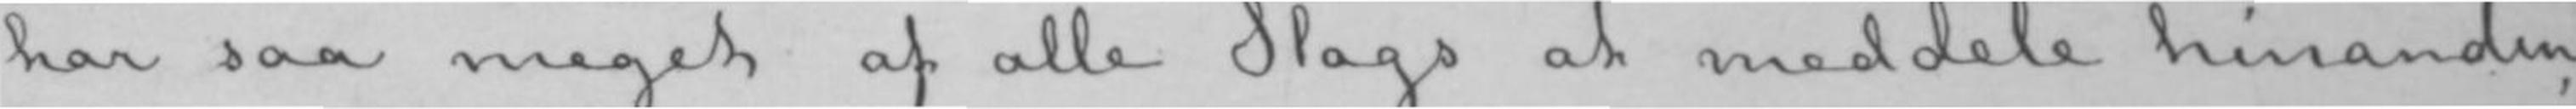har saa meget af alle Slags at meddele hinanden,
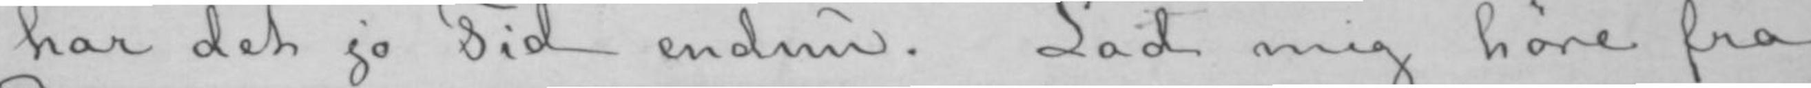har det jo Tid endnu. Lad mig høre fra
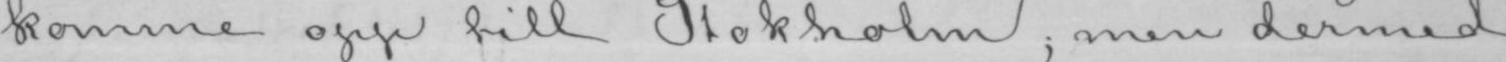komme opp till Stokholm; men dermed
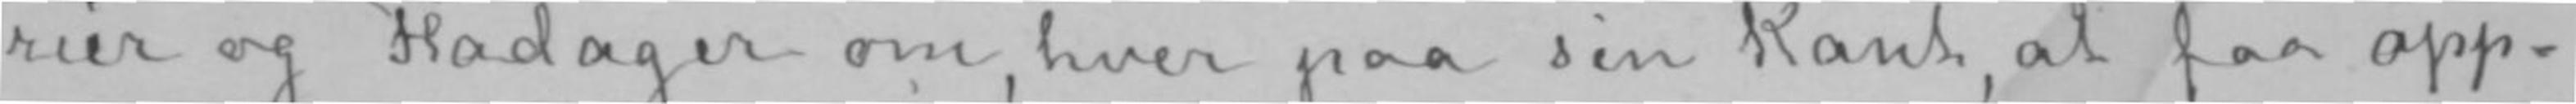rier og Fladager om, hver paa sin Kant, at faa opp-
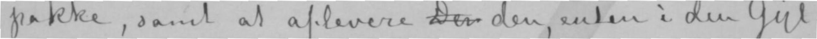pakke, samt at aflevere Den den, enten i den Gyl-
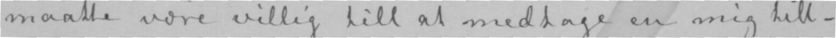maatte være villig till at medtage en mig till-
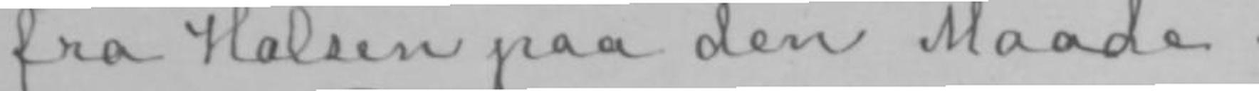fra Halsen paa den Maade.
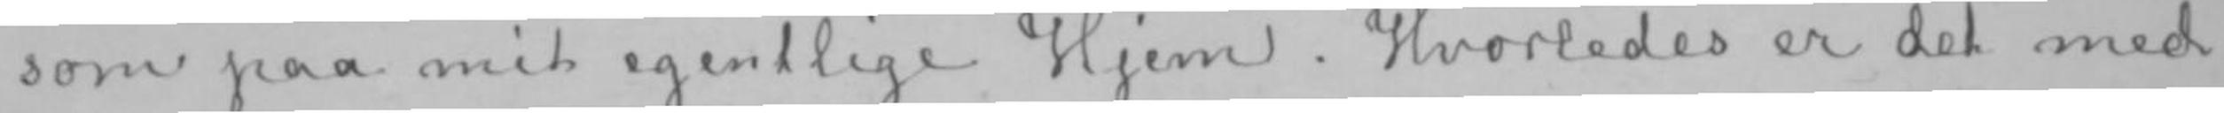som paa mit egentlige Hjem. Hvorledes er det med
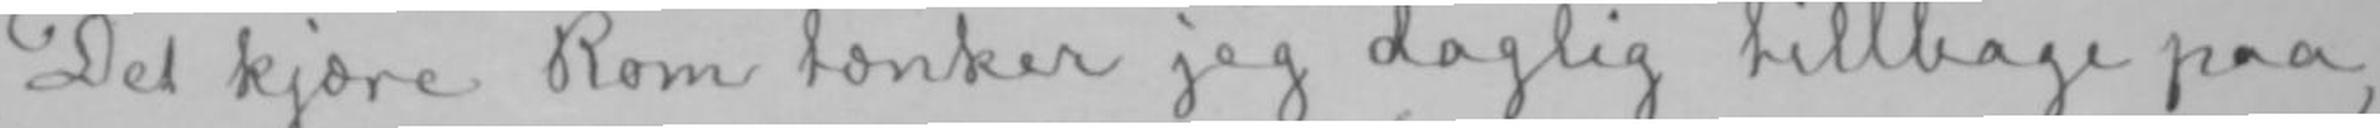Det kjære Rom tænker jeg daglig tillbage paa,
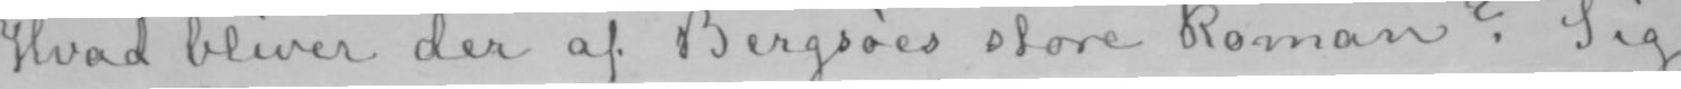Hvad bliver der af Bergsøes store Roman? Sig
